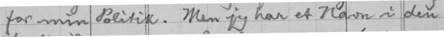for min Politik. Men jeg har et Navn i den
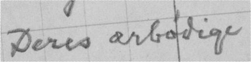Deres ærbødige
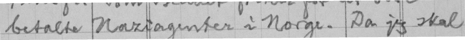betalte Naziagenter i Norge. Da jeg skal
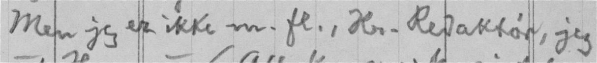Men jeg er ikke m. fl., Hr. Redaktør, jeg
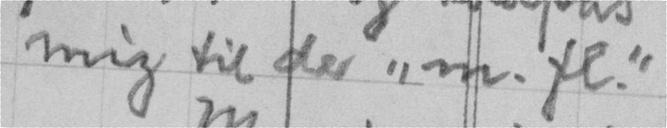mig til de "m. fl."
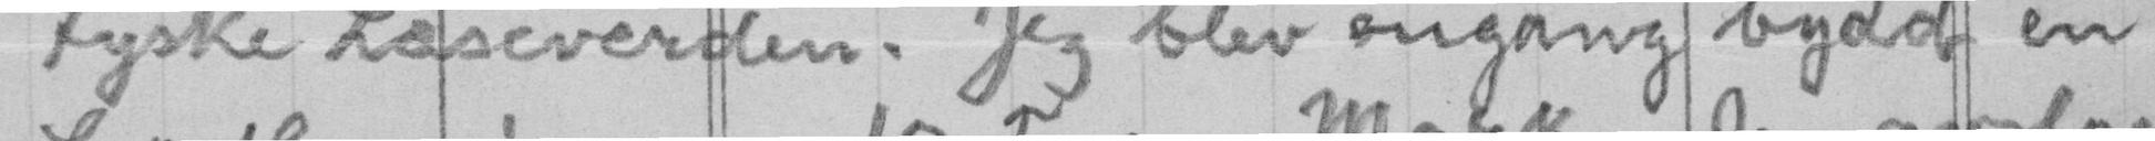tyske Læseverden. Jeg blev engang bydd en
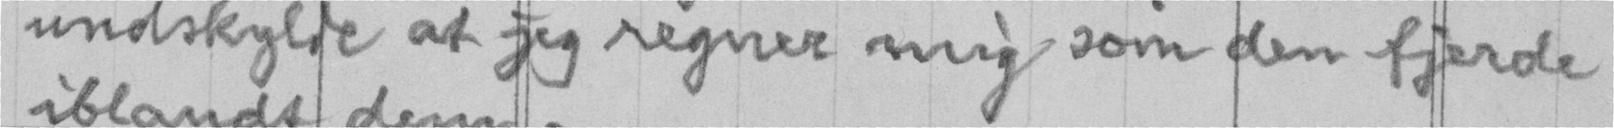undskylde at jeg regner mig som den fjerde
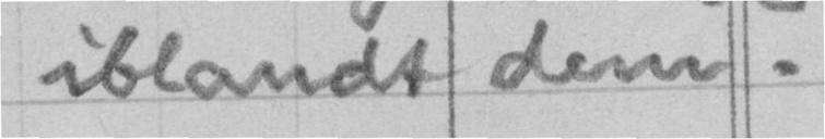iblandt dem.
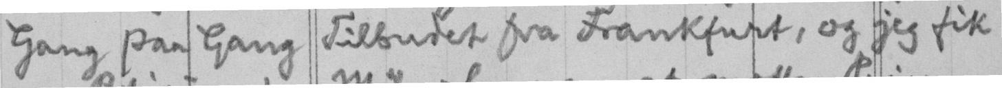Gang paa Gang Tilbudet fra Frankfurt, og jeg fik
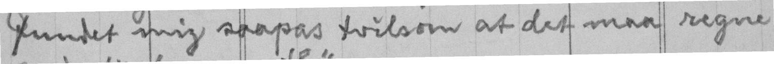fundet mig saapas tvilsom at det maa regne
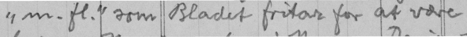"m. fl." som Bladet fritar for at være
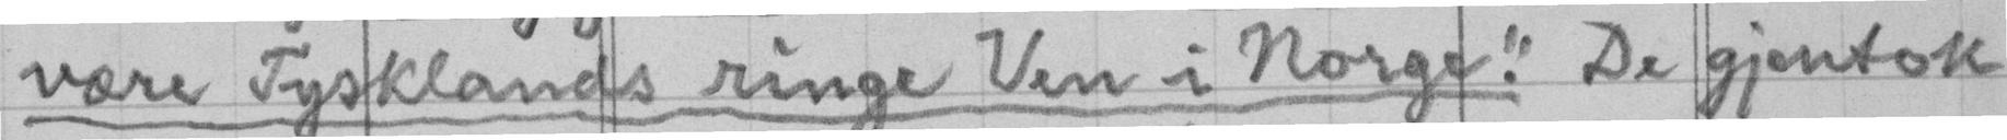være Tysklands ringe Ven i Norge." De gjentok
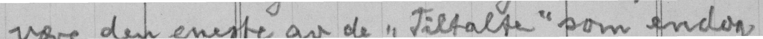være den eneste av de "Tiltalte" som endog
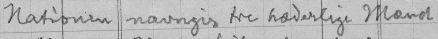Nationen navngir tre hæderlige Mænd
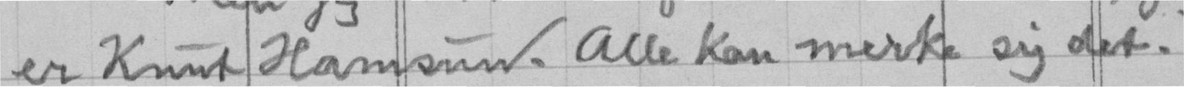er Knut Hamsun. Alle kan merke sig det.
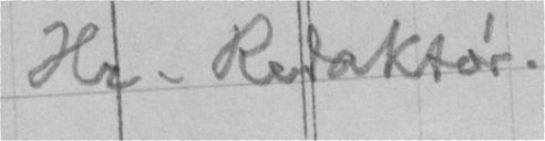Hr. Redaktør.
